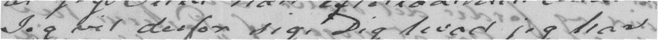Jeg vil derfor sige Dig hvad jeg har
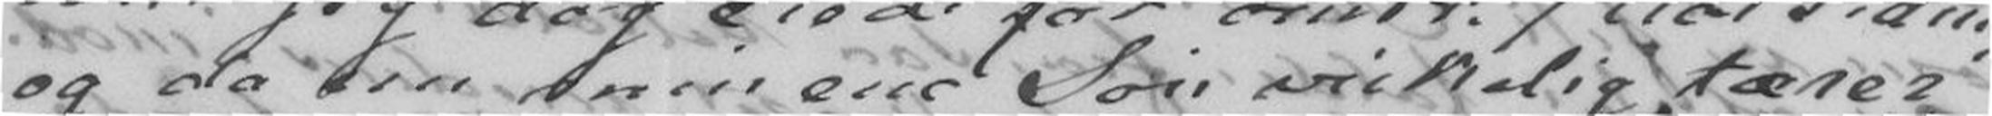og da nu min ene Søn virkelig tærer
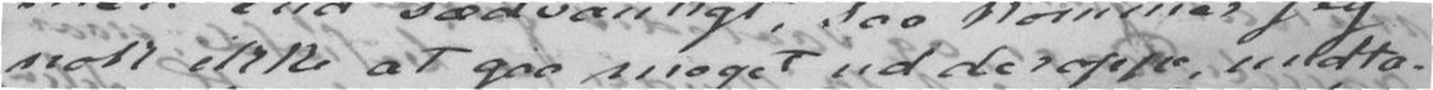nok ikke at gaa meget ud deroppe, undta-
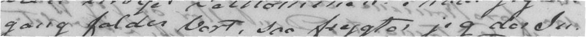gang falder bort, saa frygter jeg der In-
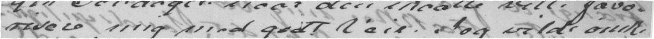risere mig med godt Veir. Jeg vilde ønske
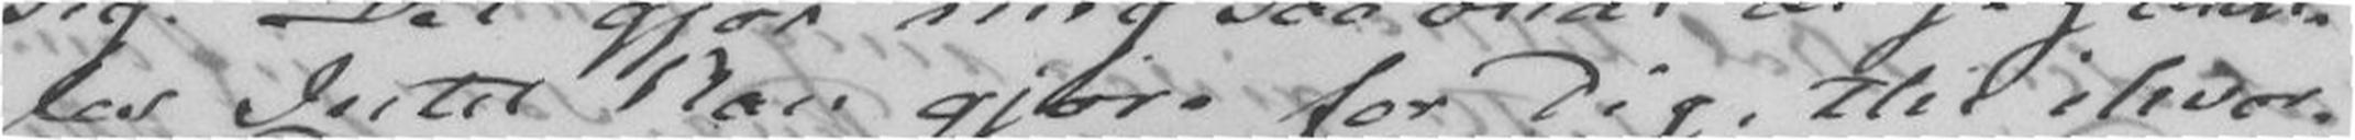les Intet kan gjøre for Dig, thi ihvor-
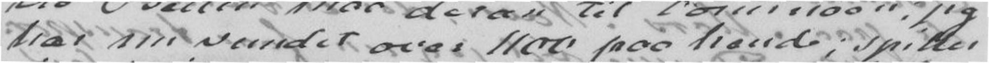har nu vundet over 1100 paa hende; spiller
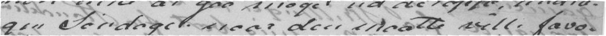gen Søndager naar den maatte ville favo-
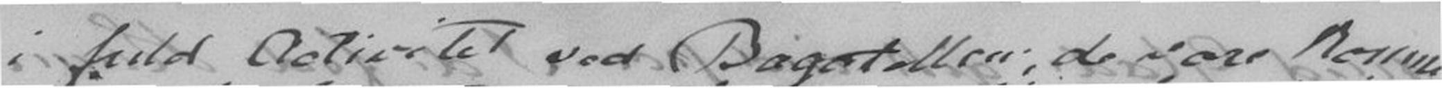i fuld Activitet ved Bagatellen, de vare komme
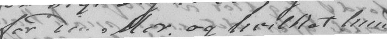foreslaaet for Din Mor, og hvilket hun
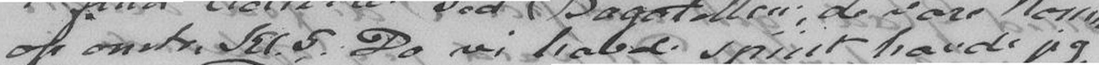op omtr. Kl.5. Da vi havde spiist havde jeg
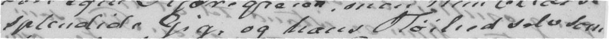splendide Gig, og hans Høihes selv som
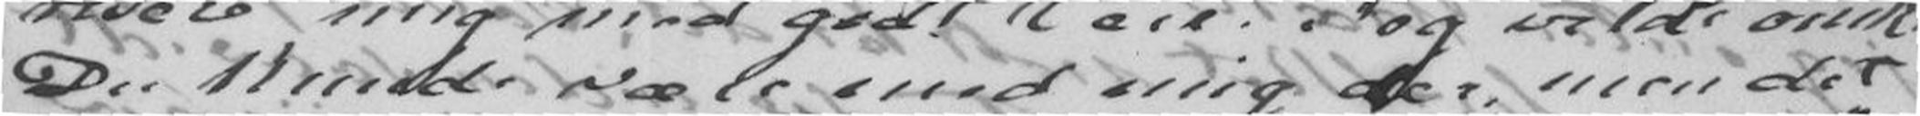Du kunde være med mig der, men det
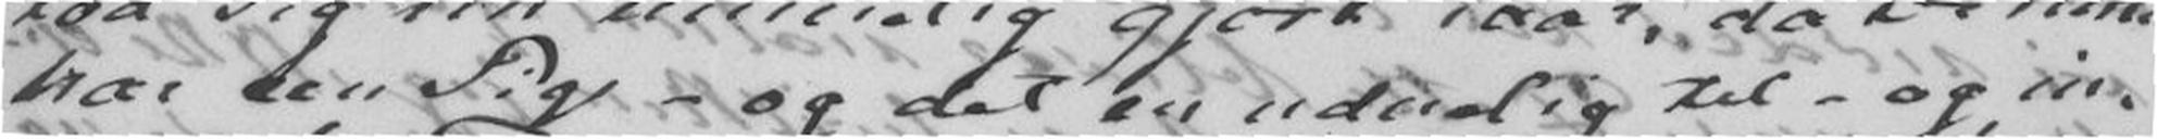har een Pige - og det en uduelig til - og in-
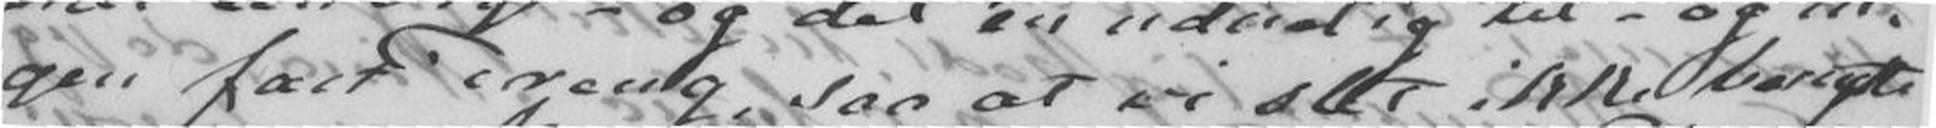gen fast Dreng, saa at vi slet ikke benytte
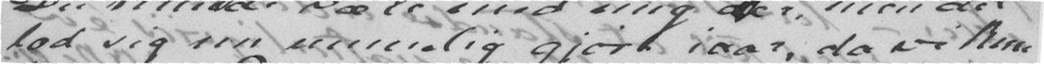lod sig nu umuelig gjøre iaar, da vi kun
